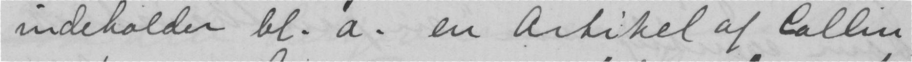indeholder bl. a. en Artikel af Collin
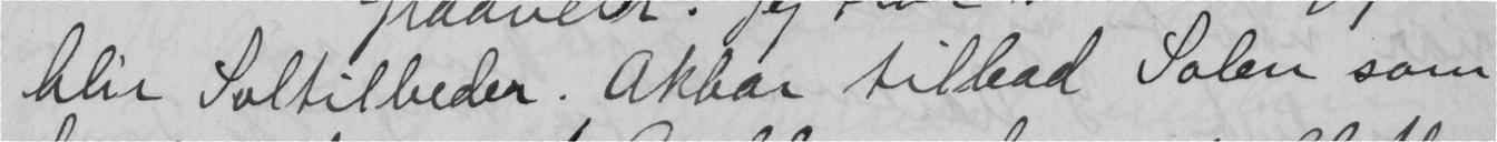blir Soltilbeder. Akbar tilbad Solen som
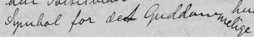Symbol for det Guddommelige
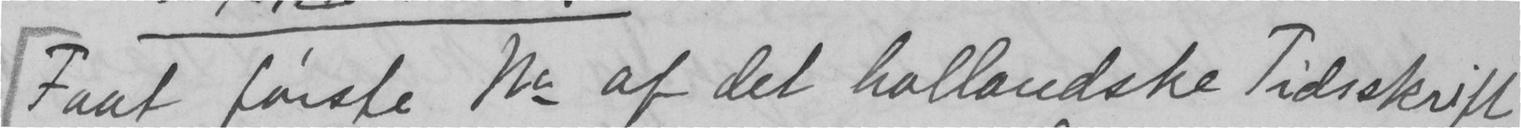Faat første No af det hollandske Tidsskift
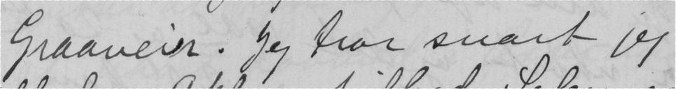Graaveir. Jeg tror snart jeg
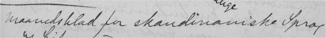maanedsblad for skandinaviske Sprog
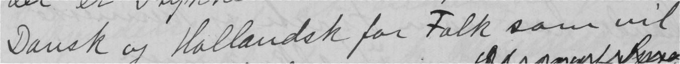Dansk og Hollandsk for Folk som vil
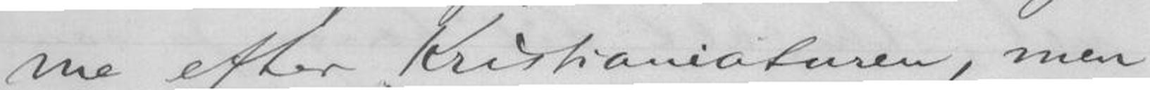me efter Kristianiaturen, men
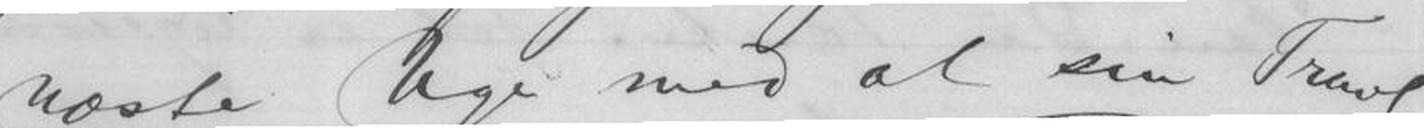næste Uge med al sin Travl-
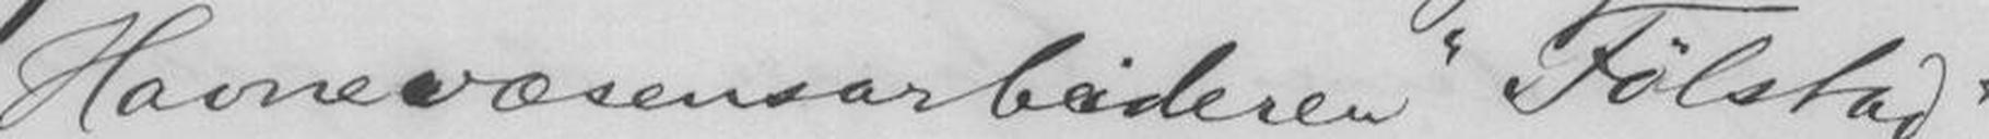Havnevæsensarbeideren "Følstad"
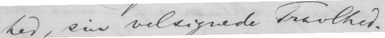hed, sin velsignede Travlhed.
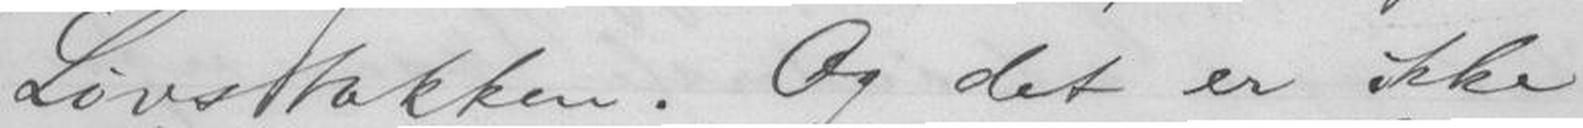Løvstakken. Og det er ikke
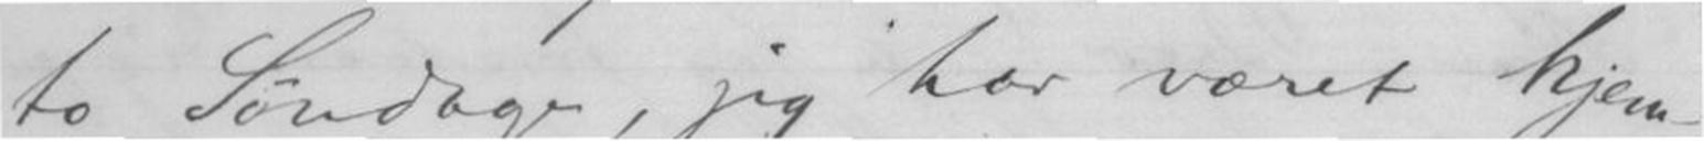to Søndage, jeg har været hjem-
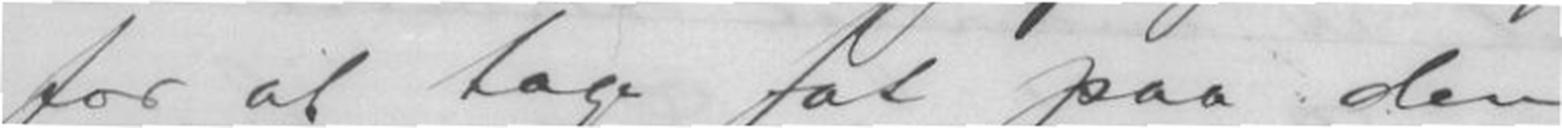for at tage fat paa den
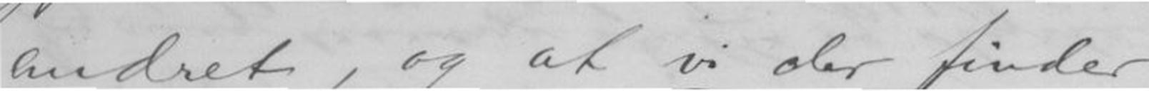andret, og at vi der finder
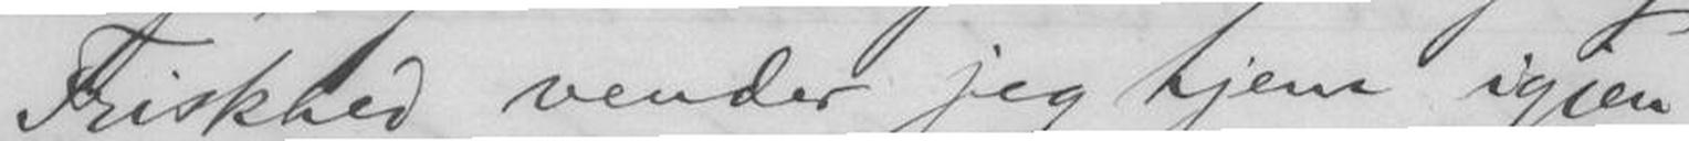Friskhed vender jeg hjem igjen
